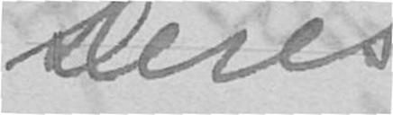Deres
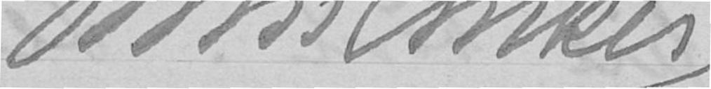Nini Anker
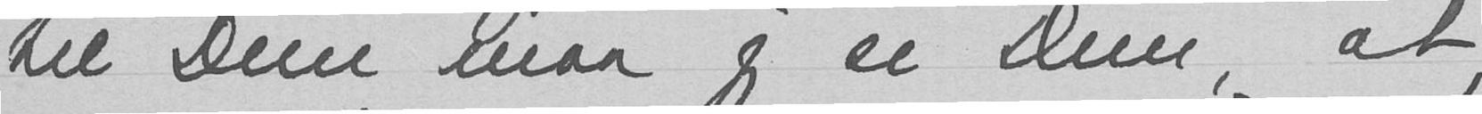til Dem maa jeg si Dem, at
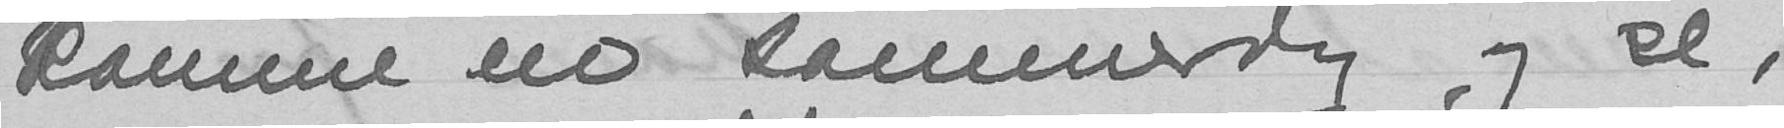komme en sommerdag og se,
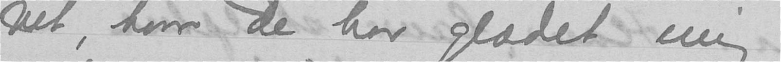vey, hvor De har glædet mig
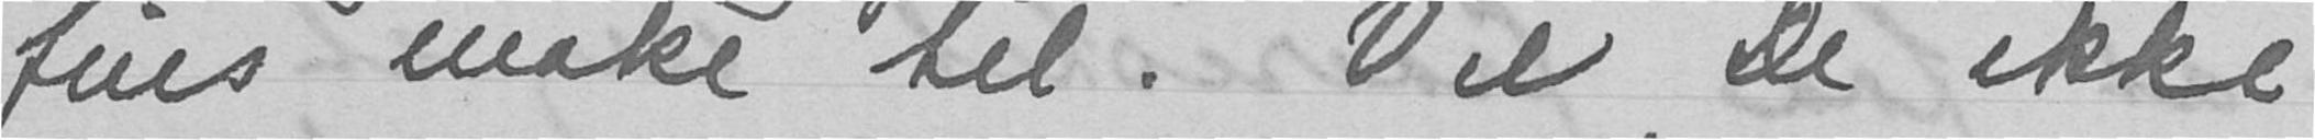fins make til. Vil De ikke
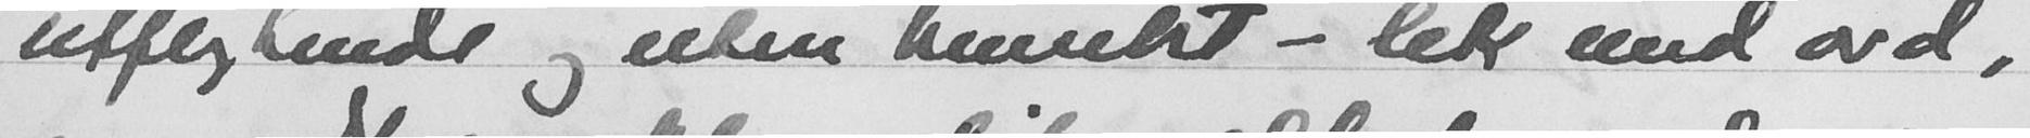utflytende og uten benrikt - lek med ord,
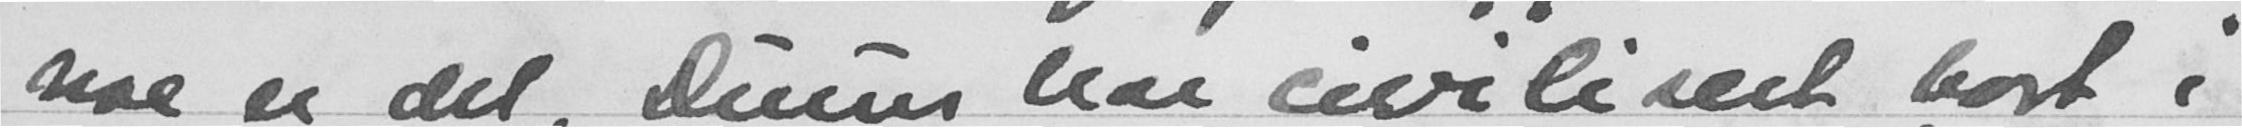noe er det, Duun har civilisert bort i
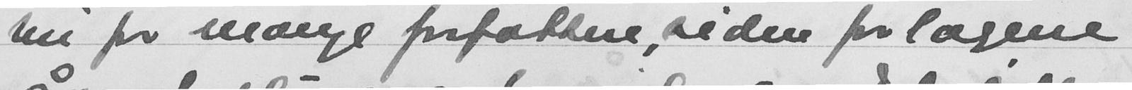vei for mange forfattere, siden forlagene
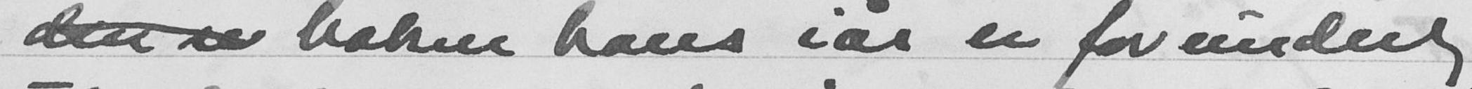boken hans iår er forunderlig
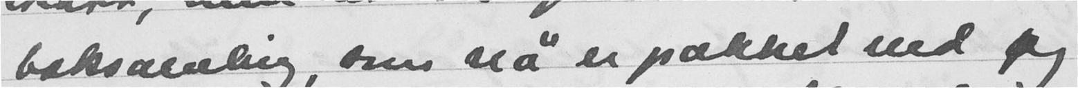boksamling, som nå er pakket ind og
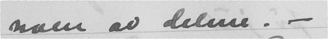noen av delene. -
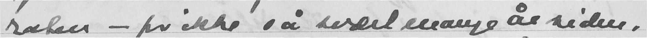ratur - for ikke så sværtmange år siden.
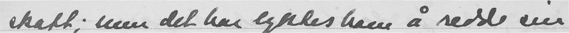skatt; men det har lyktes ham å redde sin
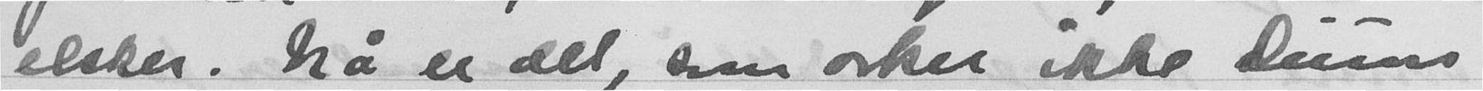elsker. Nå er det, som orker ikke Duun
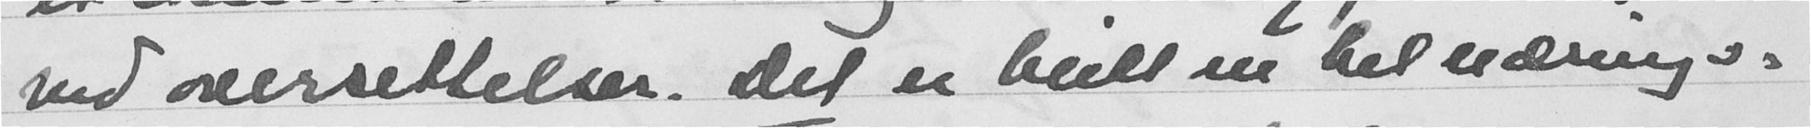med oversettelser. Det er blitt en hel nærings-
